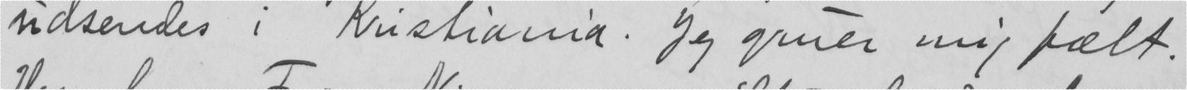udsendes i Kristiania. Jeg gruer mig fælt.
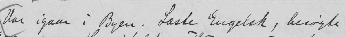Var igaar i Byen. Læste Engelsk, besøgte
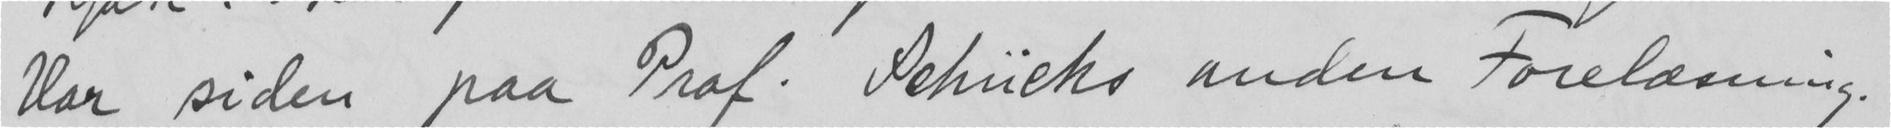Var siden paa Prof. Schücks anden Forelæsning.
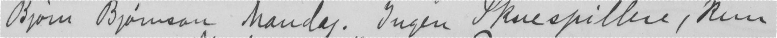Bjørn Bjørnson Mandag. Ingen Skuespillere, kun
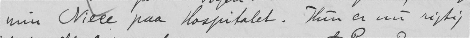min Niece paa Hospitalet. Hun er nu rigtig
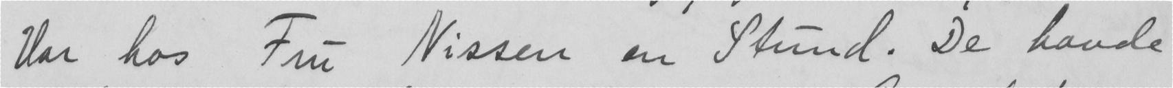Var hos Fru Nissen en Stund. De havde
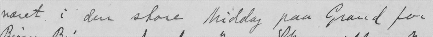været i den store Middag paa Grand for
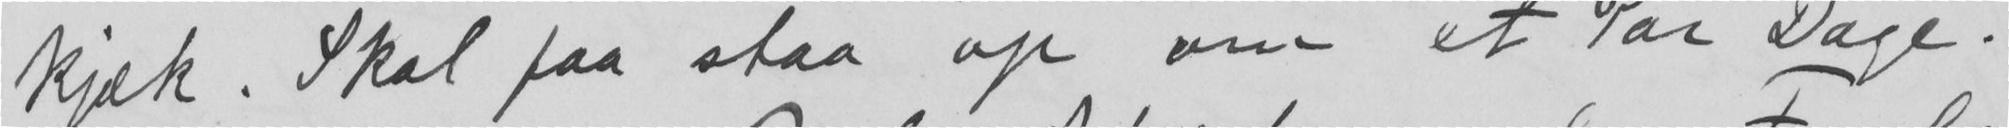kjæk. Skal faa staa op om et Par Dage.
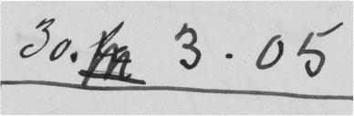30. 3.05
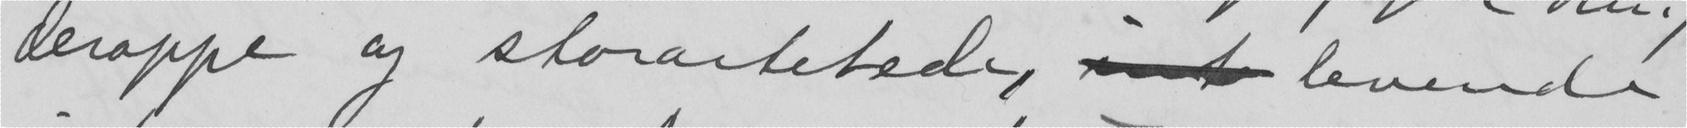deroppe og storartetede  levende
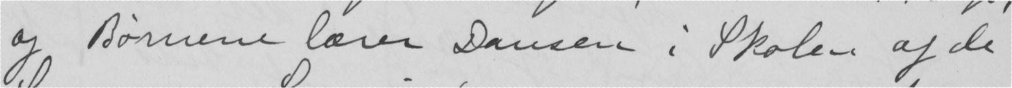og Børnene lærer Dansen i Skolen af de
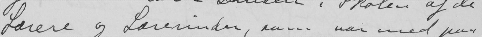Lærere og Lærerinder, som var med paa
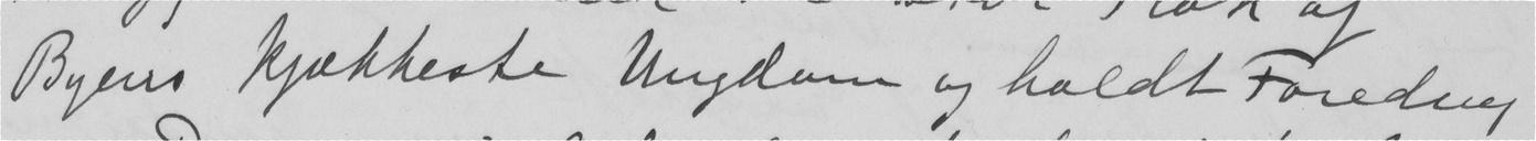Byens kjækkeste Ungdom og holdt Foredrag
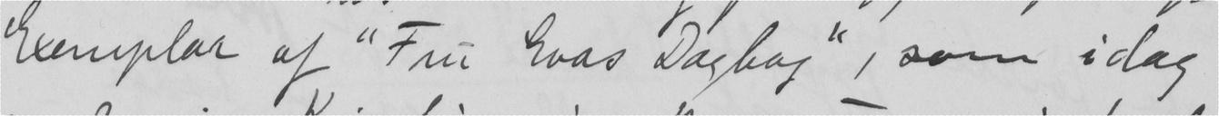Exemplar af "Fru Evas Dagbog", som idag
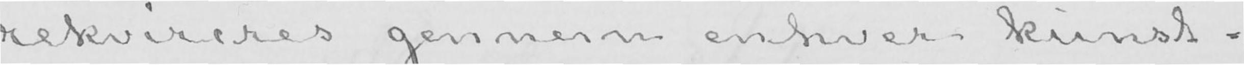rekvireres gennem enhver kunst-
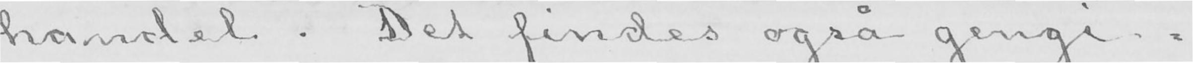handel. Det findes også gengi-
